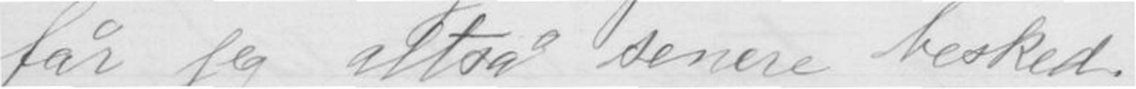får jeg altså senere besked.
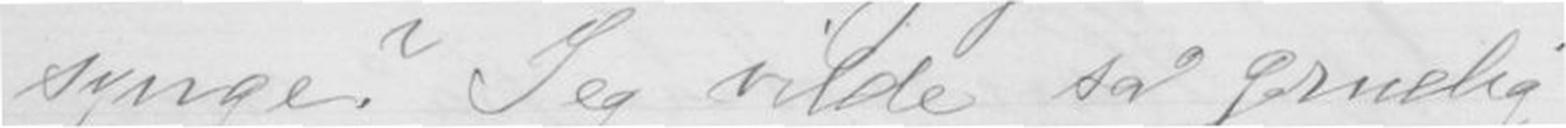synge? Jeg vilde så gruelig
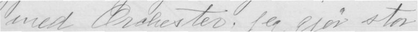med Orkester; jeg gjør stor
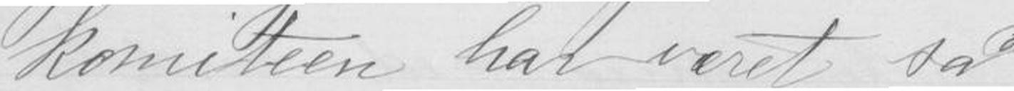komiteen har været så
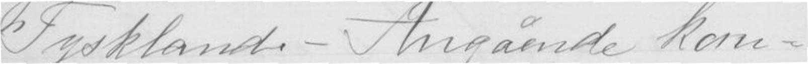Tyskland.- Angående kom-
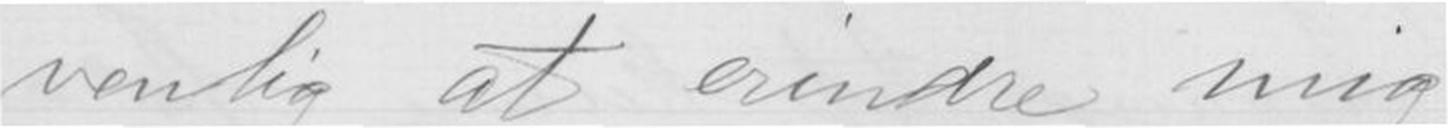venlig at erindre mig,
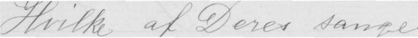Hvilke af Deres sange
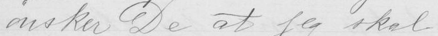ønsker De at jeg skal
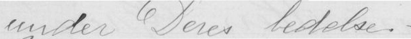under Deres ledelse.
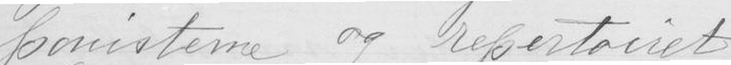ponisterne og repertoiret
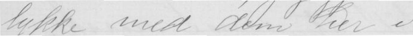lykke med dem her i
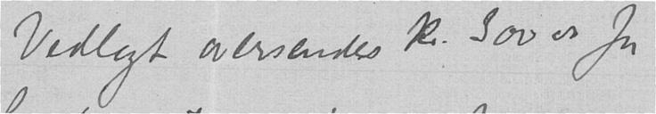Vedlagt oversendes kr. 300 00 for
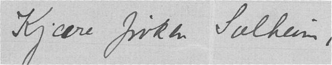Kjære frøken Solheim,
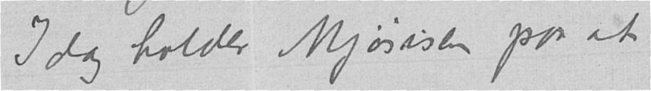I dag holder Mjøsisen paa at
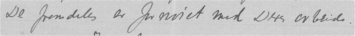De fremdeles er fornøiet med Deres arbeide.
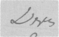Deres
